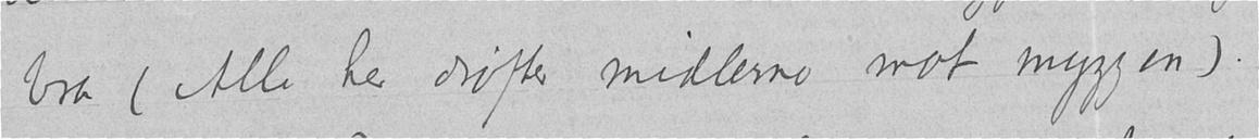bra (Alle her drøfter midlerne mot myggen).
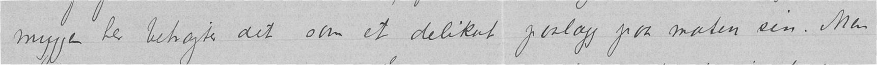myggen her betragter det som et delikat paalægg paa maten sin. Men
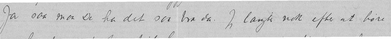Ja saa maa De ha det saa bra da. Jeg længter nok efter at høre
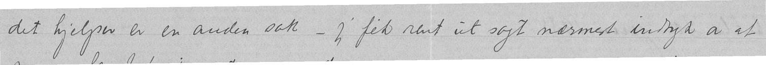det hjelper er en anden sak – jeg fik rent ut sagt nærmest indtryk av at
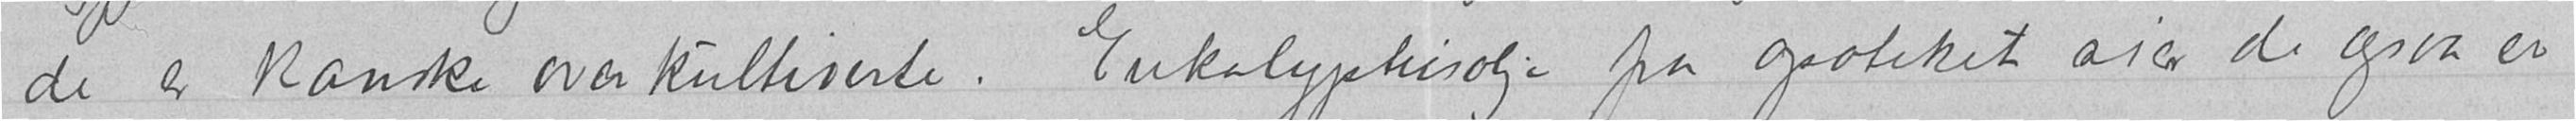de er kanske overkultiverte. Eukalyptusolje fra apoteket sier de ogsaa er
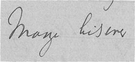Mange hilsener
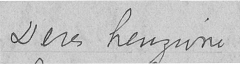Deres hengivne
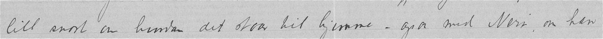litt snart om hvordan det staar til hjemme – ogsaa med Neri, om han
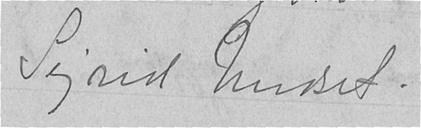Sigrid Undset.
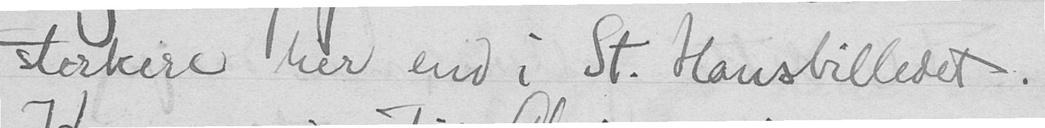sterkere her end i St. Hansbilledet.
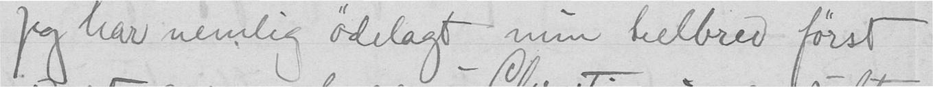Jeg har nemlig ødelagt min helbred først
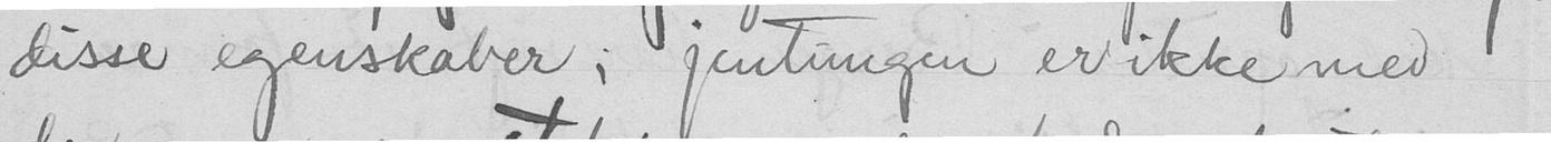disse egenskaber; jentungen er ikke med
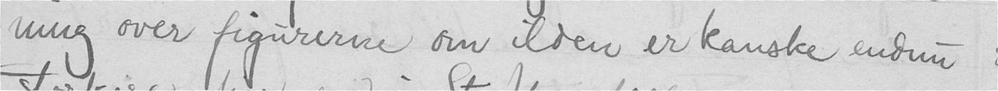tng over figurerne om ilden er kanske endnu
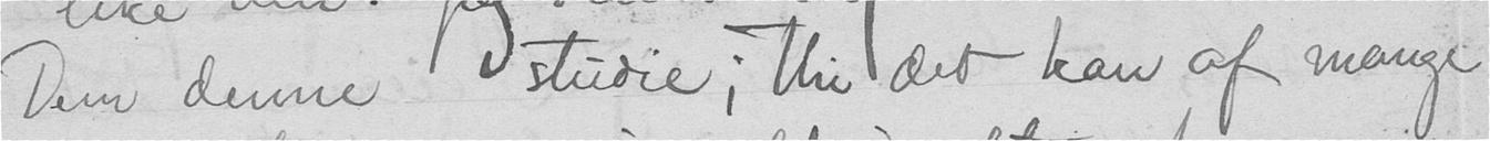Dem denne studie; thi det kan af mange
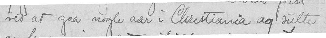ved at gaa nogle aar i Christiania og sulte
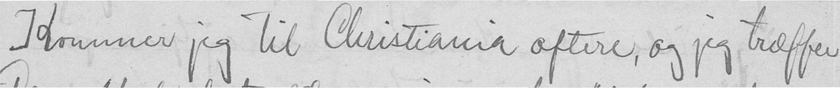Kommer jeg til Cristiania oftere, og jeg træffer
